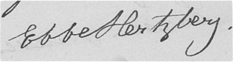Ebbe Hertzberg.
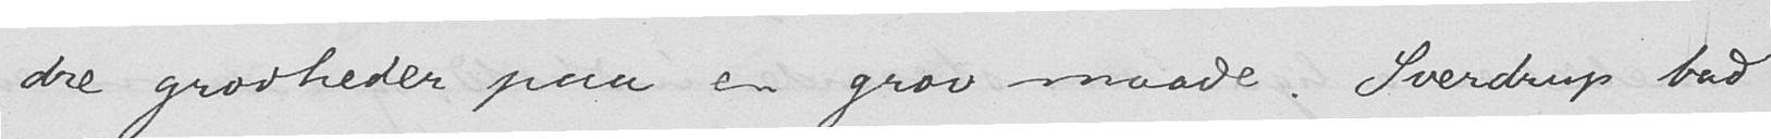dre grovheder paa en grov maade. Sverdrup bad
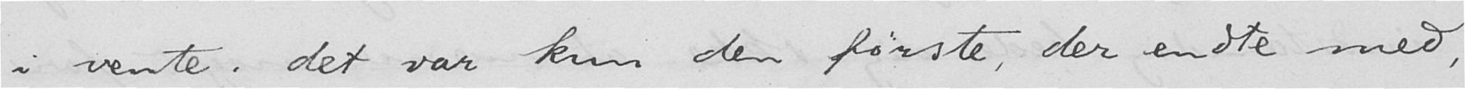i vente; det var kun den første, der endte med,
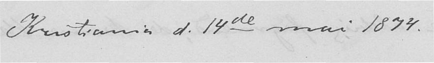Kristiania d. 14de mai 1874.
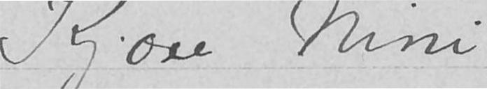Kjære Nini,
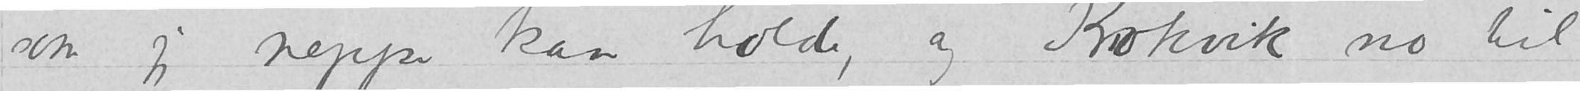som jeg neppe kan holde, og Krokvik no til
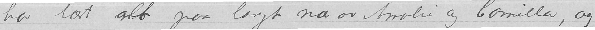har læst alt paa langt nær av Amalie og Camilla, og
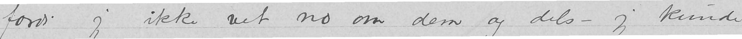fordi jeg ikke vet no om dem og dels – jeg kunde
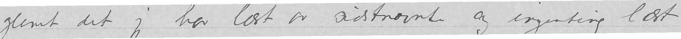glemt det jeg har læst av sistnævnte og ingenting læst
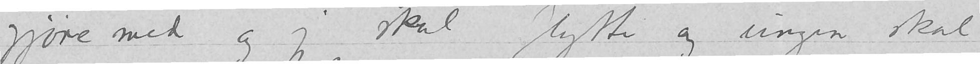gjøre med og jeg skal flytte og ungen skal
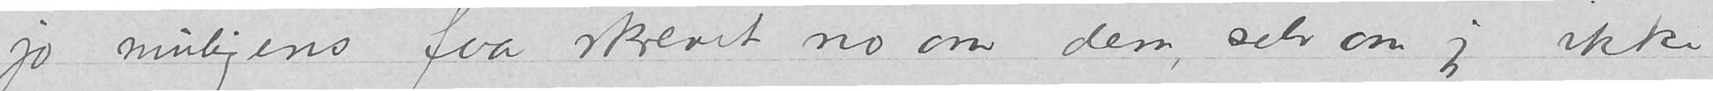jo muligens faa skrevet no om dem, selv om jeg ikke
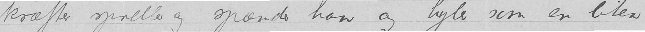kræfter spreller og spænder han og hyler som en liten
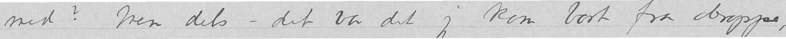med? Men dels – det var det jeg kom bort fra deroppe,
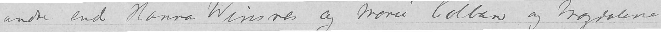andre end Hanna Winsnes og Marie Colban og Magdalene
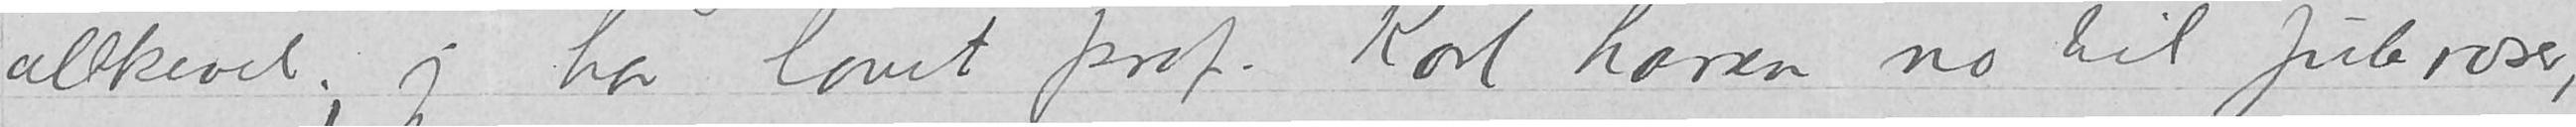allikevel; jeg har lovet prof. Karl Larsen no til Juleroser,
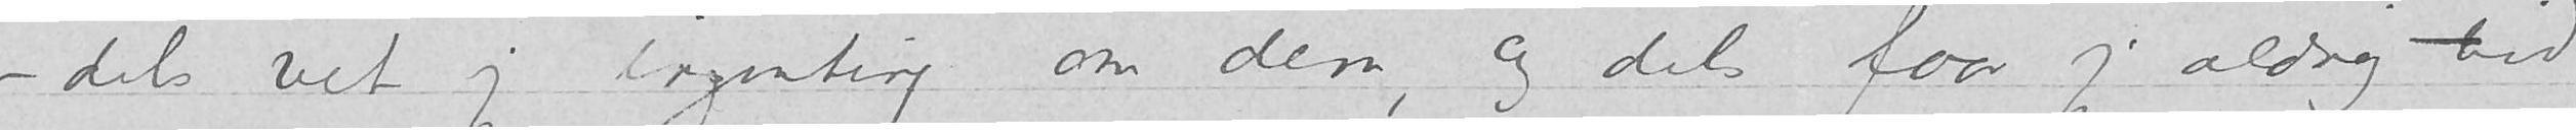– dels vet jeg ingenting om dem, og dels faar jeg aldri tid
